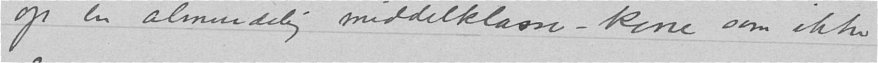op en almindelig middelklasse-kone som ikke
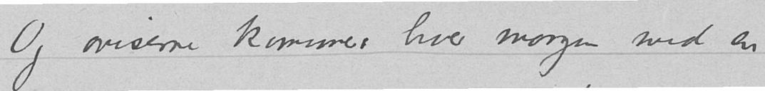Og aviserne kommer hver morgen med en
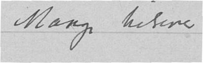Mange hilsener
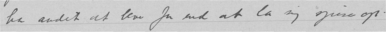har andet at leve for end at la sig spise op.
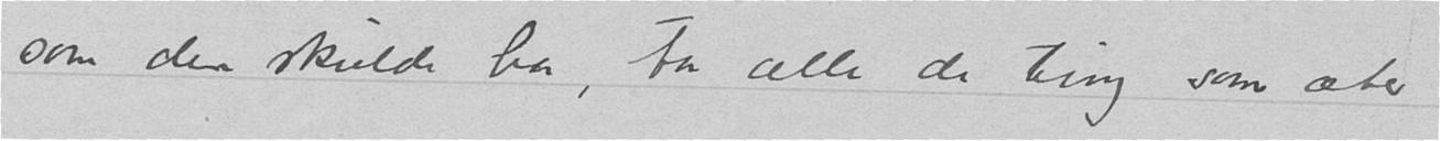som den skulde ha, ta alle de ting som æter
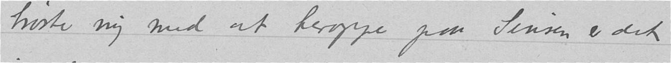trøste mig med at heroppe paa Sinsen er det
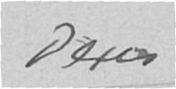Deres
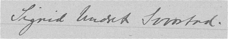Sigrid Undset Svarstad.
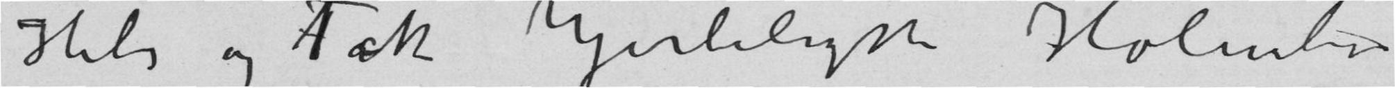Hils og Ttak hjerteligst Holmboe
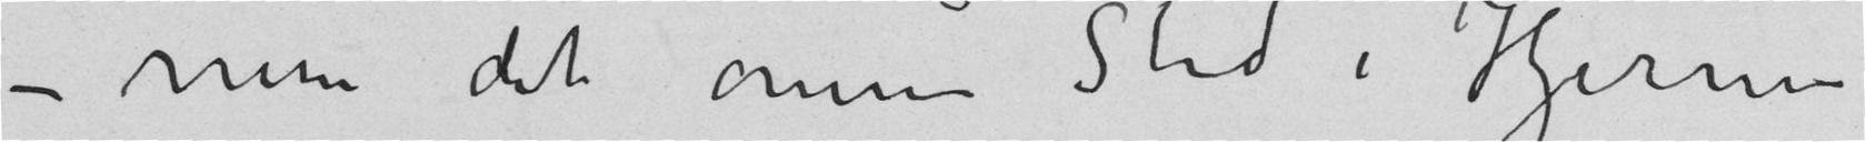– Men det ømme Sted i Hjernen
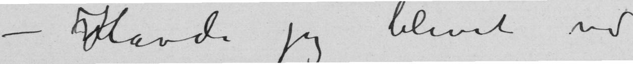– Havde jeg blevet ved
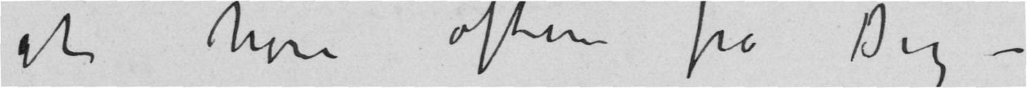at høre oftere fra dig –
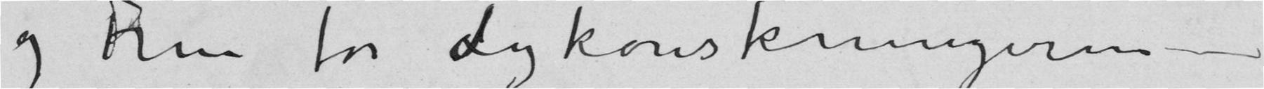og Frue for Lykønskningerne –
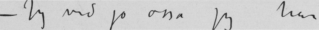– Jeg ved jo osså jeg har
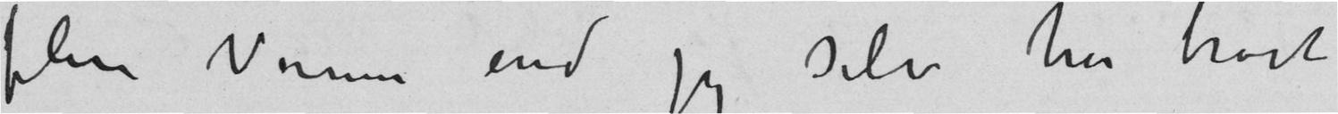flere Venner end jeg selv har troet
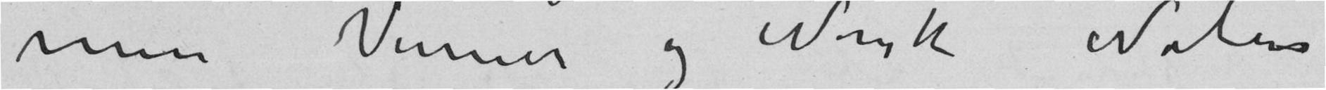mine Venner og Norsk Natur
